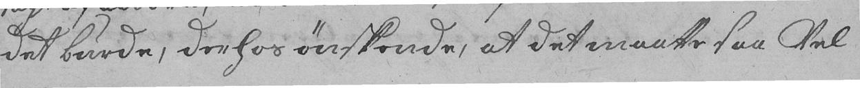det burde, der hos ønskende, at det maatte saa Vel
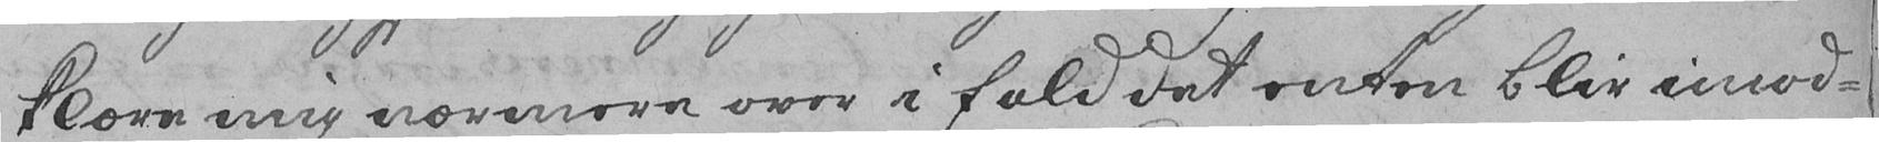klære mig nærmere over i fald det enten blir imod-
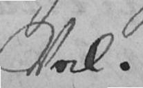Vel.
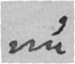nu
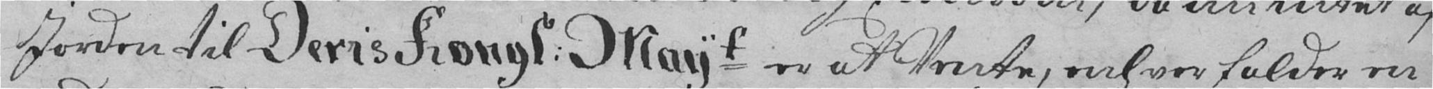Jorden til Deris Kongl. Mayt er at Vente, enhver falder en
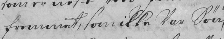fremmet, som ikke Var Søn
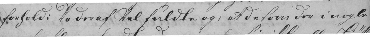forhold. Da deraf Vel fuldte og, at de som der i nogle
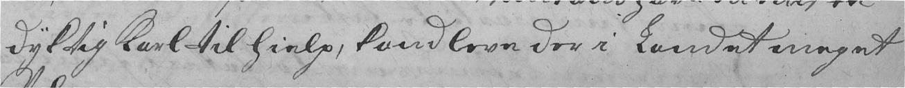dyktig Karl til hielp, kand leve der i Landet meget
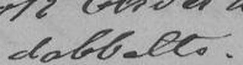dobbelte.
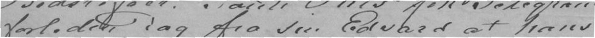forleden Dag fra sin Edvard at hans
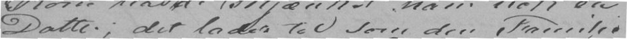Datter; det lades til som den Familie
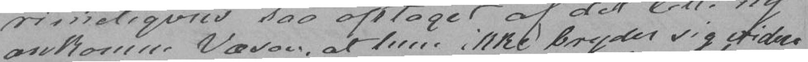ankomme Væsen, at hun ikke bryder sig videre
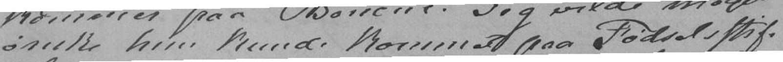ønske hun kunde komme paa Fødselstif-
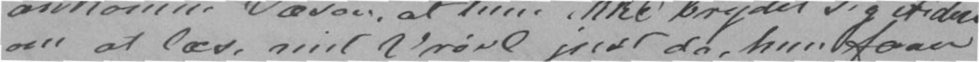om at læse mit Vrøvl just da, hun faaer
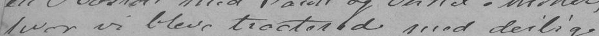hvor vi bleve tractered med deilig
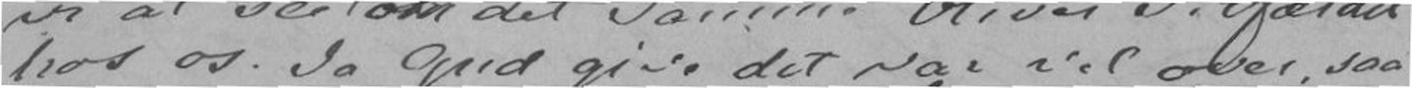hos os. Ja Gud give det var over, saa
